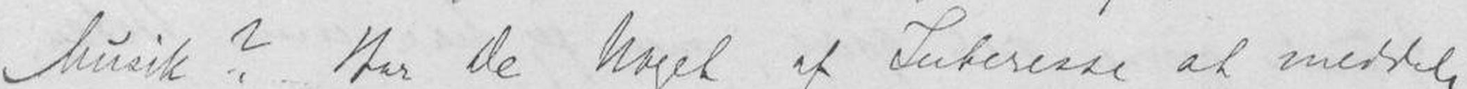Musik? Har de noget af Interesse af meddele
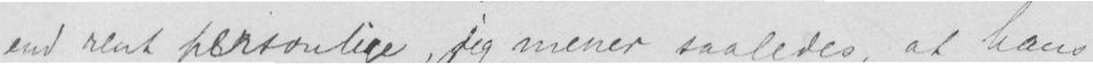end rent pærsonlige, jeg mener saaledes, at hans
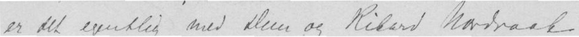er det egentlig med Dem og Rikard Nordraak
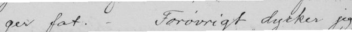ger fat. - Forøvrigt dyrker jeg
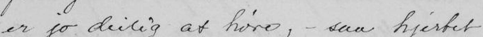er jo deilig at høre, - saa hjertet
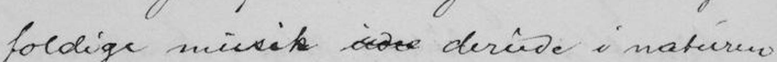foldige musik derinde i naturens
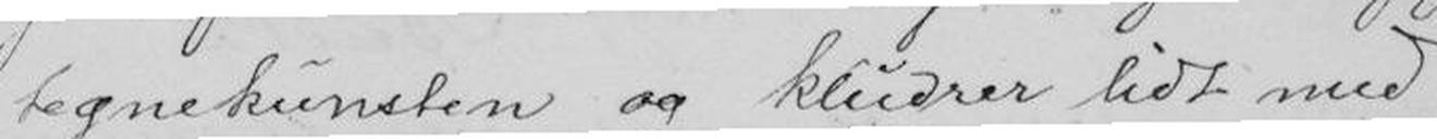tegnekunsten og kludrer lidt med
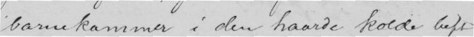barnekammer i den haarde kolde luft,
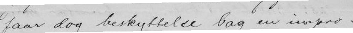faar dog beskyttelse bag en impro-
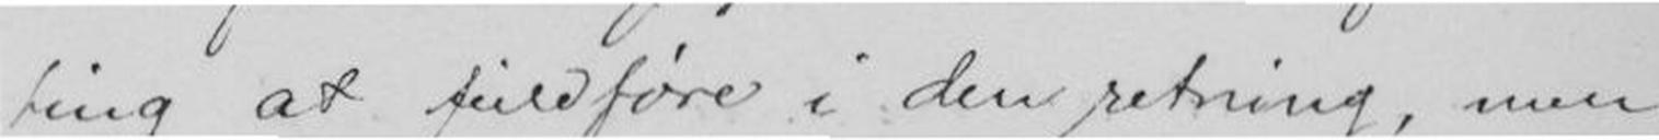ting at fuldføre i den retning, men
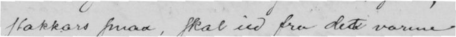stakkars smaa, skal ud fra det varme
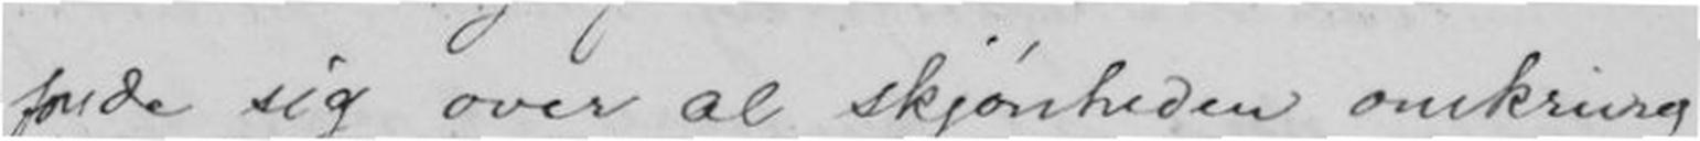fryde sig over al skjønheden omkring
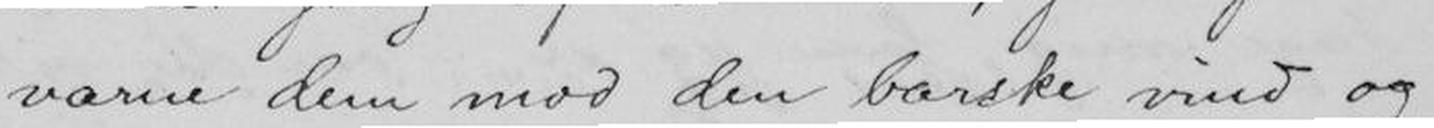varme dem mod den barske vind og
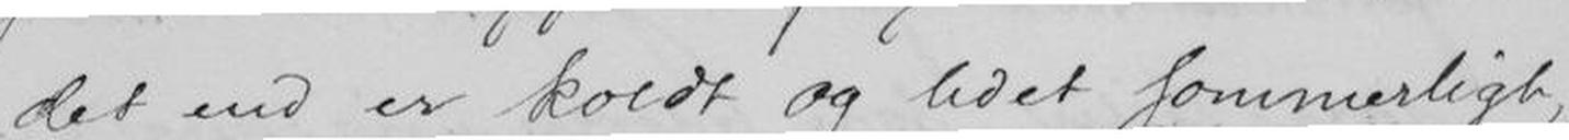det end er koldt og lidet sommerligt,
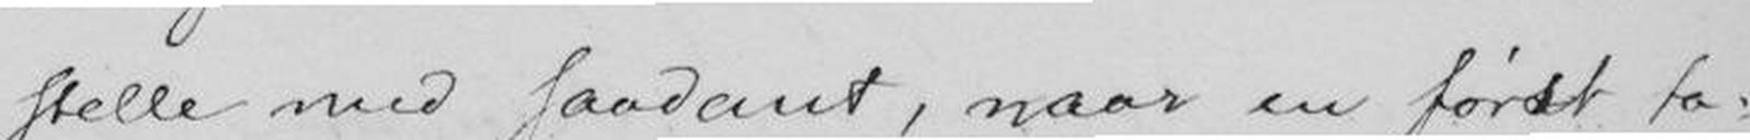stelle med saadant, naar en først ta-
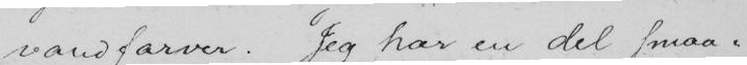vandfarver. Jeg har en del smaa-
